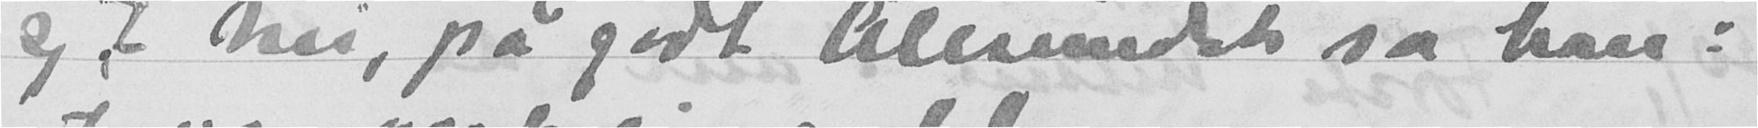og hei, på godt Ålesundsk sa han:
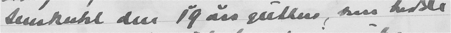Lemkuhl den 19 års gutten, som hadde
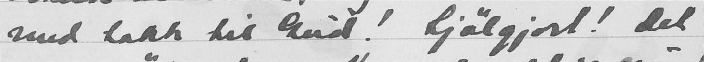med takk til Gud! Sjølgjort! det
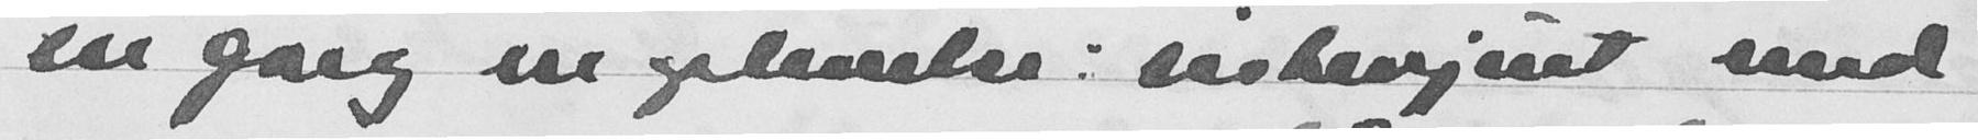en gang en oplevelse; intervjuet med
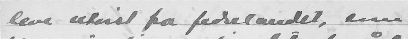leve utvist fra fedrelandet, som
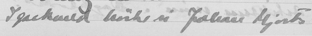Igårkveld hørte vi Johan Hjorts,
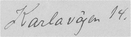Karlavägen 14,
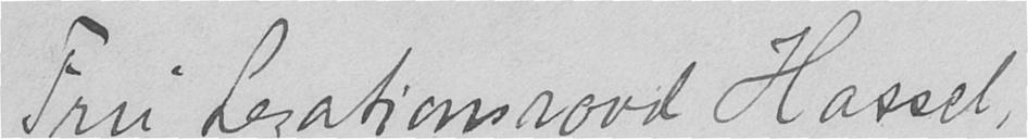Fru Legationsraad Hassel,
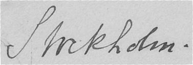Stockholm.
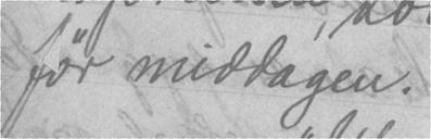før middagen.
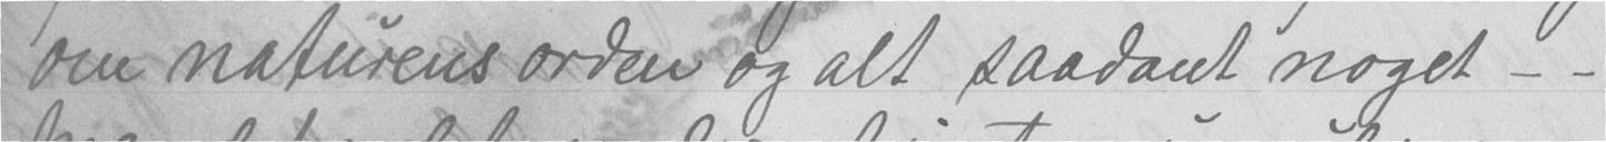om naturens orden og alt saadant noget - -
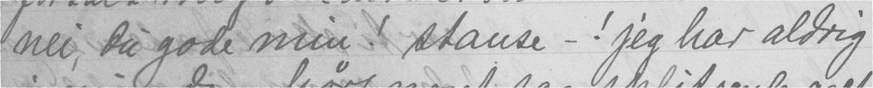nei, du gode min! stanse - ! jeg har aldrig
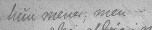hun mener; men -
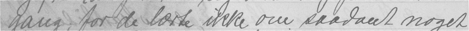gang; for de lærte ikke om saadant noget
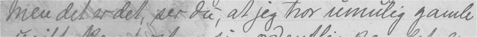Men det er det, ser du, at jeg tror umulig gamle
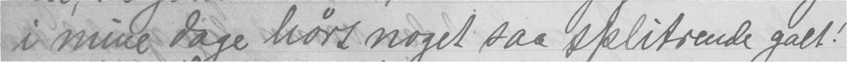i mine dage hørt noget saa splitrende galt!"
